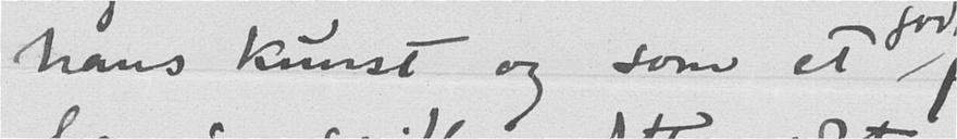hans kunst og som et
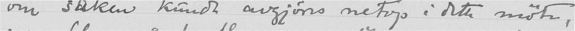om saken kunde avgjøres netop i dette møte,
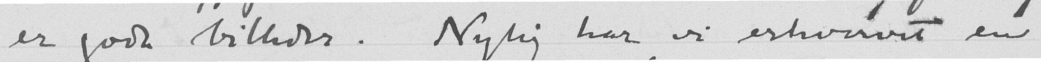er gode billeder. Nylig har vi erhvervet en
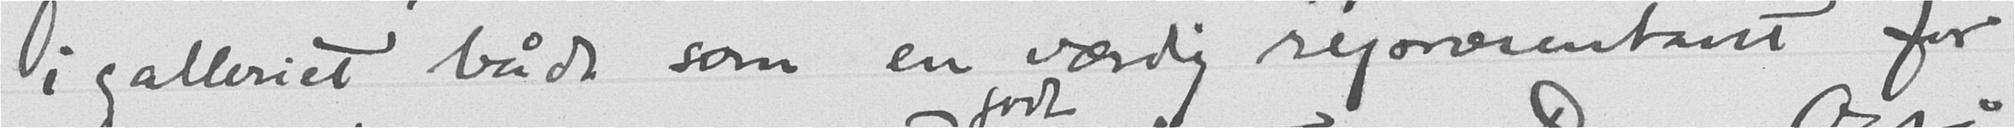i galleriet både som en værdig repræsentant for
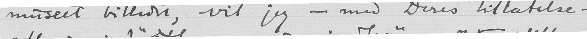museet billedet, vil jeg - med Deres tillatelse -
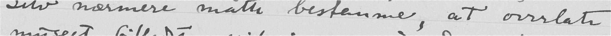selv nærmere måtte bestemme, at overlate
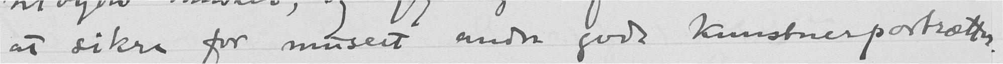at sikre for museet andre gode kunstnerportrætter.
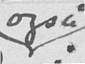også
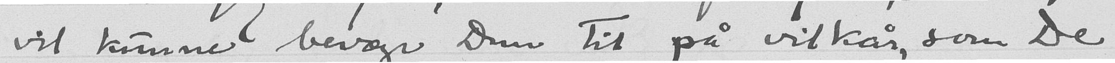vil kunnet bevæge Dem til på vilkår, som De
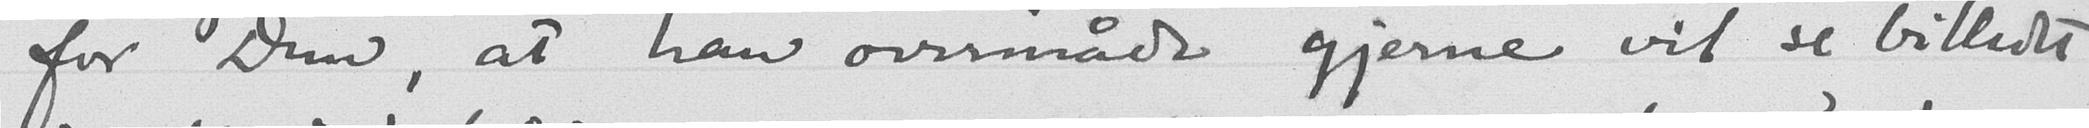for Dem, at han overmåde gjerne vil se billedet
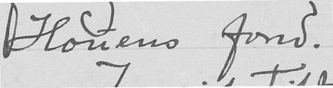Houens fond.
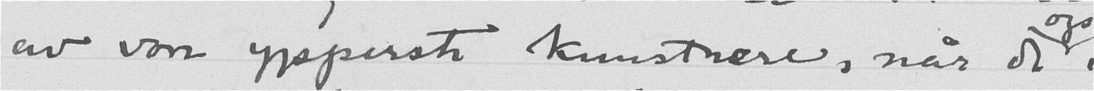av vore ypperste kunstnere, når de
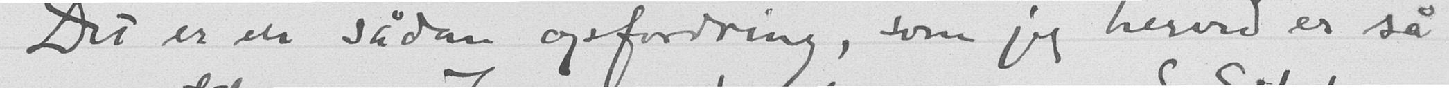Det er en sådan opfordring, som jeg herved er så
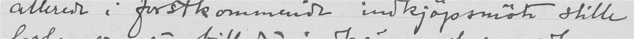allerede i førstkommende indkjøpsmøte stille
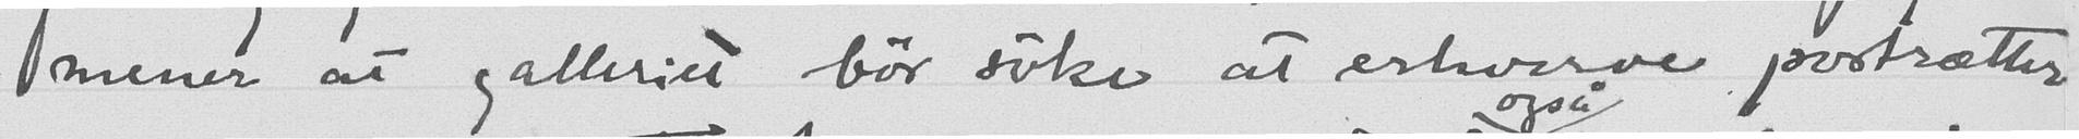mener at galleriet bør søke at erhverve postrætter
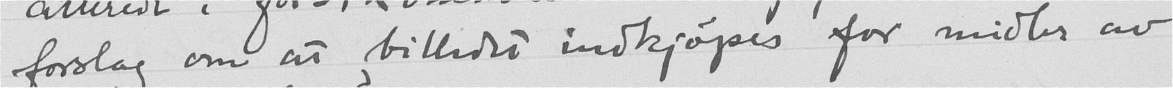forslag om at billedet indkjøpes for midler av
